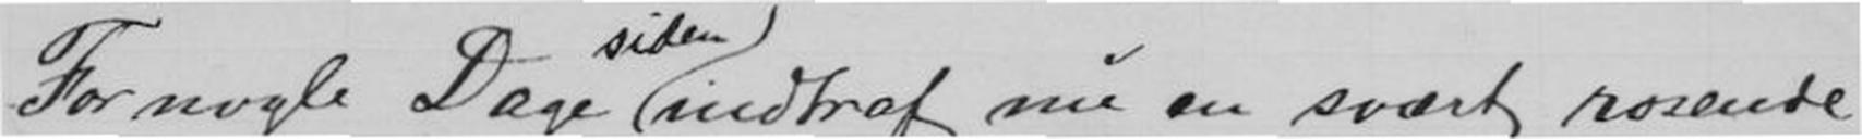For nogle Dage indtraf nu en svært rosende
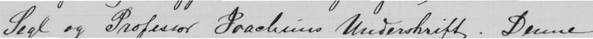Segl og Professor Joachims Underskrift. Denne
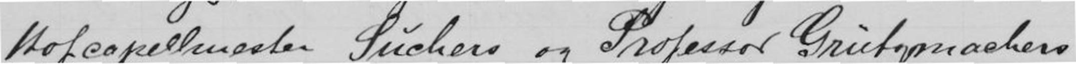Hofcapellmecher Suchers og Professor Grützmachers
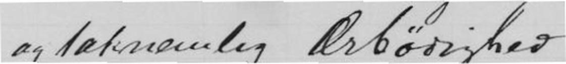og taknemlig Ærbødighed
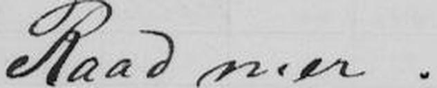Raad mer. -
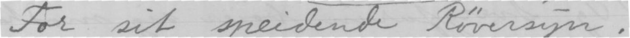For sit speidende Røversyn.
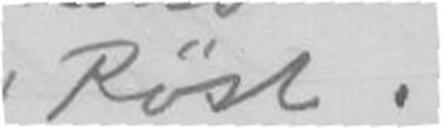Røst.
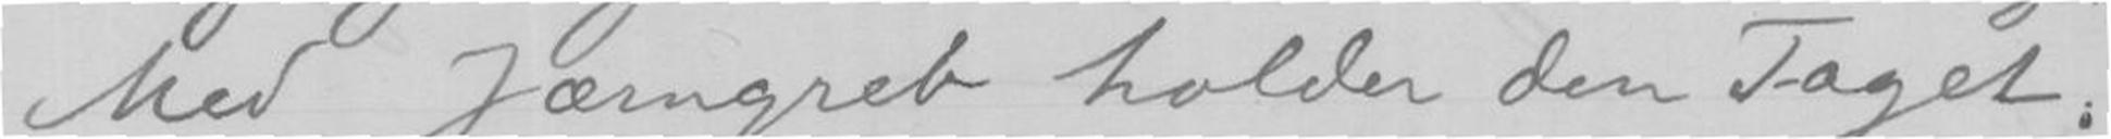Med jærngreb holder den Taget.
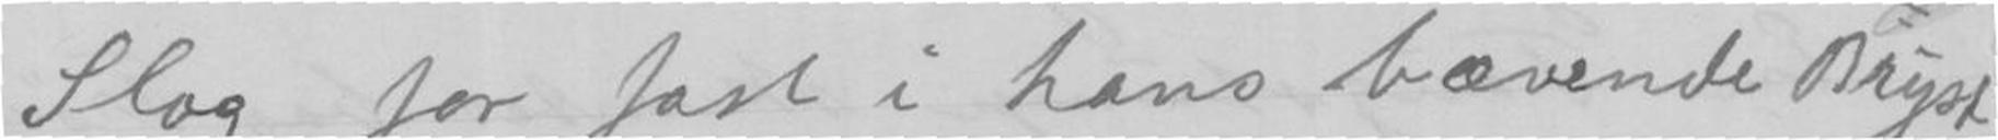Slog for fast i hans bævende Bryst.
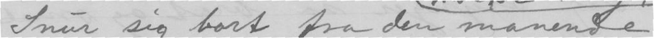Snur sig bort fra den manende
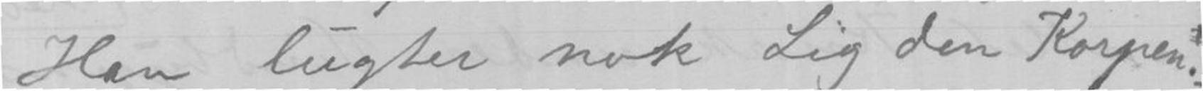Han lugter nok Lig den Korpen.
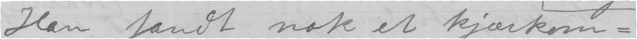Han fandt nok et kjærkom
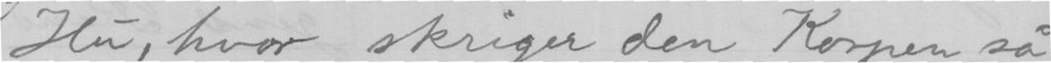Hu, hvor skriger den Korpen så
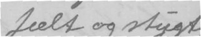fælt og stygt,
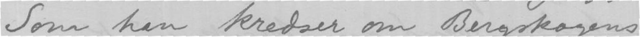Som han kredser om Bergskogens
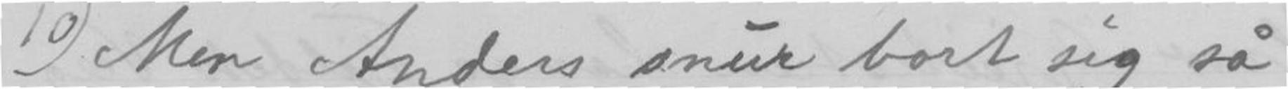Men Anders snur bort sig så tung
